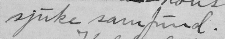sjuke samfund.
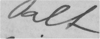alt
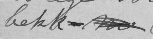bekk -.
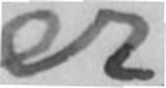er
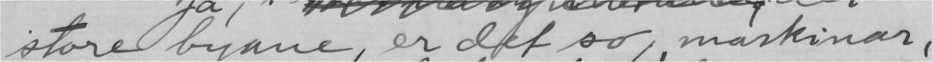store byane, er det so, maskinar,
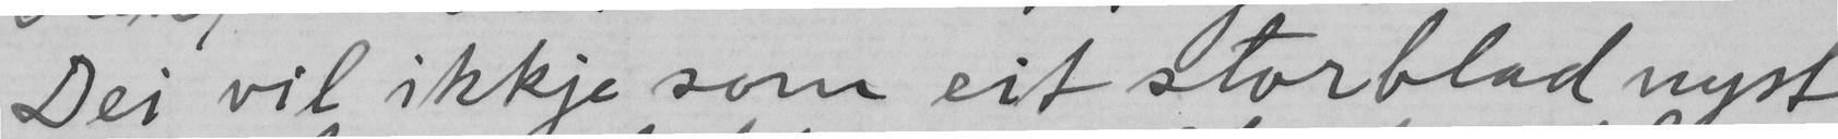Dei vil ikkje som eit storblad nyst
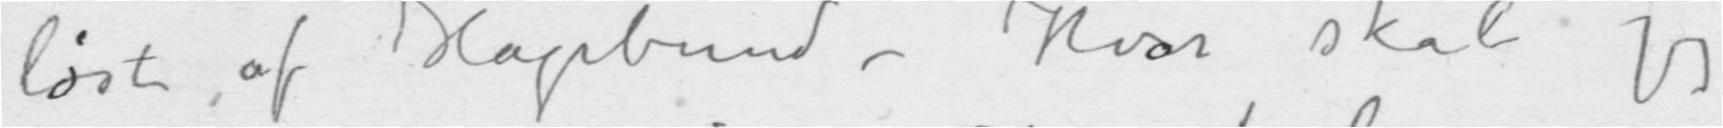løst af Hagebund – Hvor skal jeg
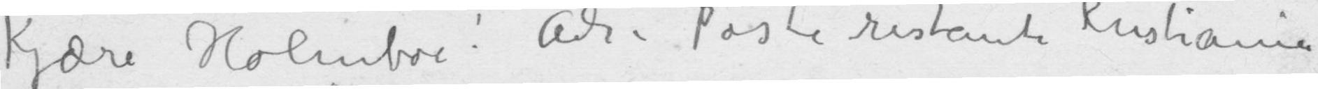Kjære Holmboe! Adr. Poste restante Kristiania
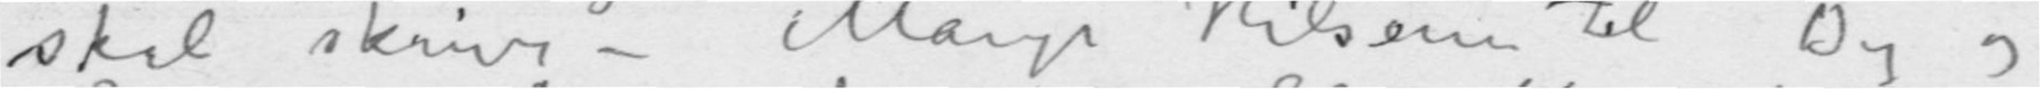skal skrive –Mange Hilsener til Dig og
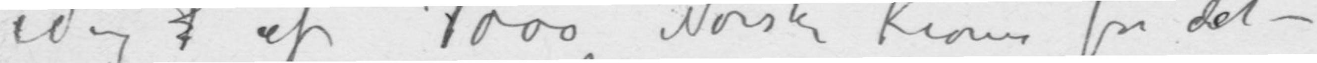idagaf 7000 Norske kroner for det –
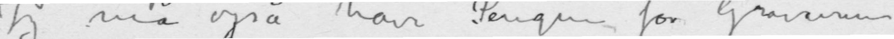Jeg må også have Pengene for Gravurene
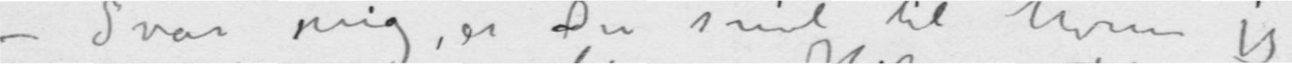– Svar mig, er Du snil til hvem jeg
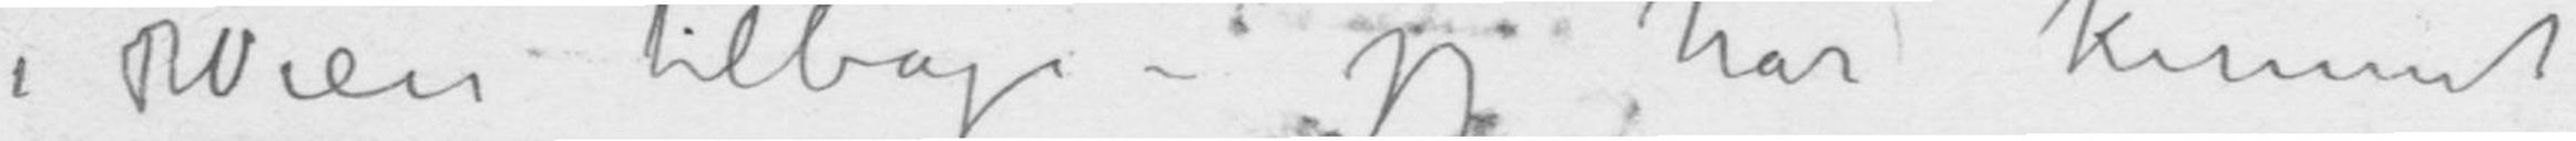i Wien tilbage – jeg har kunnet
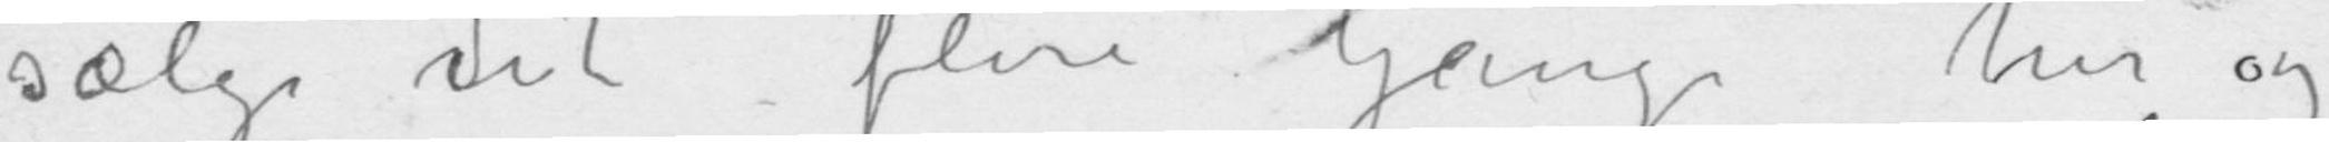sælge det flere Gange her og
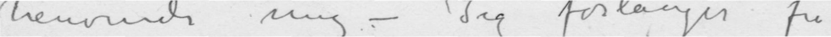henvende mig – Jeg forlanger fra
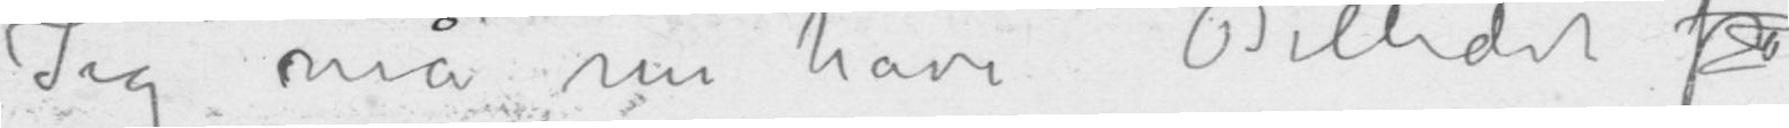Jeg må nu have Billedet fra
